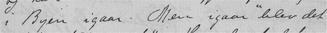i Byen igaar. Men igaar "blev det
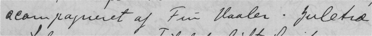acompagneret af Fru Vaaler. Juletræ
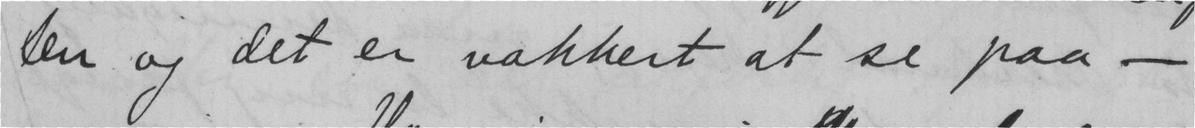ten og det er vakkert at se paa -
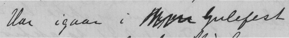Var igaar i  julefest
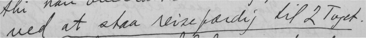ved at staa reisefærdig til 2 Toget.
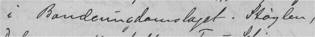i Bondeungdomslaget. Støylen,
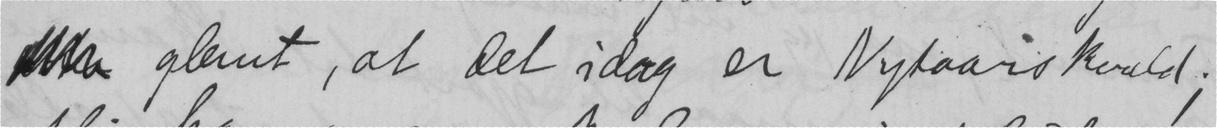glemt, at det idag er Nytaarskvæld;
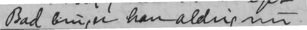Bad bruger han aldrig nu
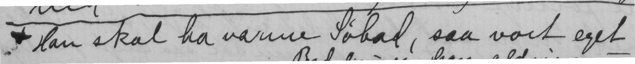x Han skal ha varme Søbad, saa vort eget
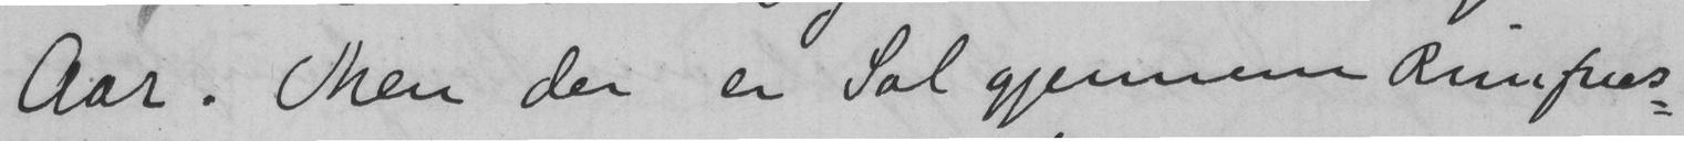aar. Men der er Sol gjennem Rimfros-
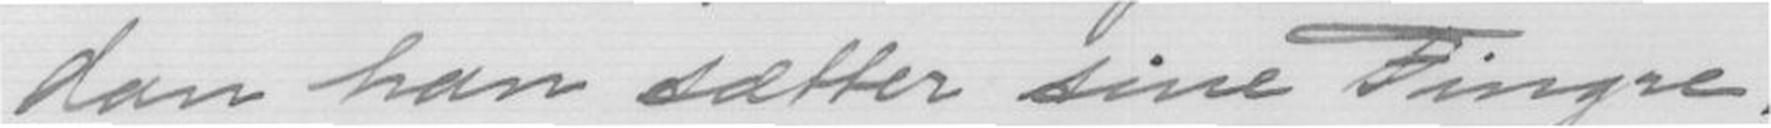dan han sætter sine Fingre.
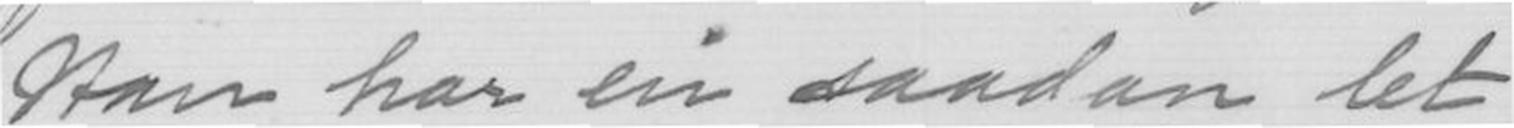Man har en saadan let
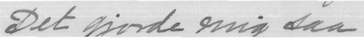Det gjorde mig saa
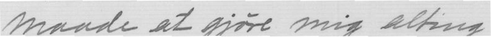maade at gjøre mig alting
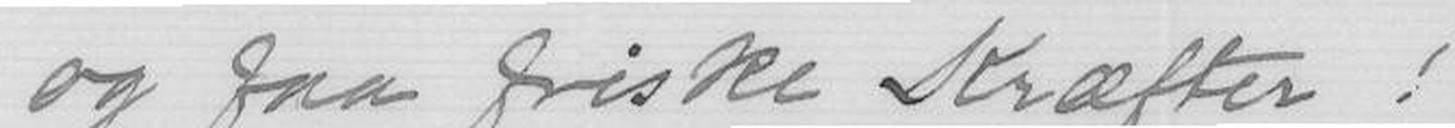og faa friske Kræfter!
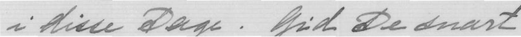i disse Dage. Gid De snart
